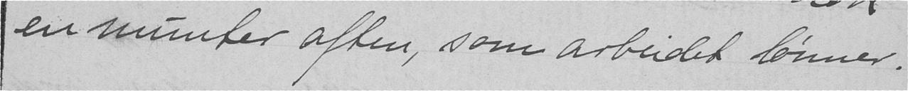en munter aften, som arbeidet lønner.
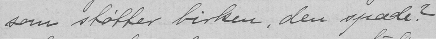som støtter birken, den spæde?
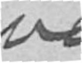vel
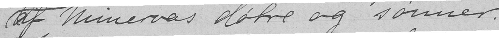af Minervas døtre og sønner.
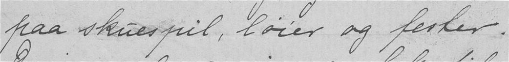paa skuespil, løier og fester.
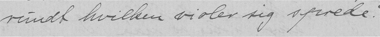rundt hvilken violer sig sprede?
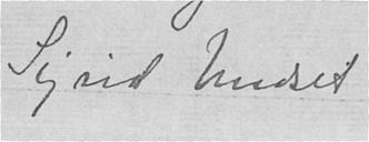Sigrid Undset
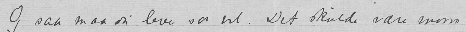Og saa maa du leve saa vel. Det skulde være morro
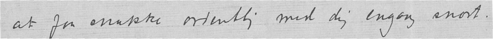at faa snakke ordentlig med dig engang snart.
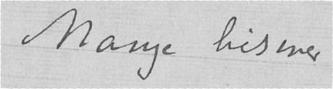Mange hilsener
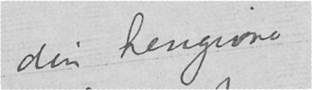din hengivne
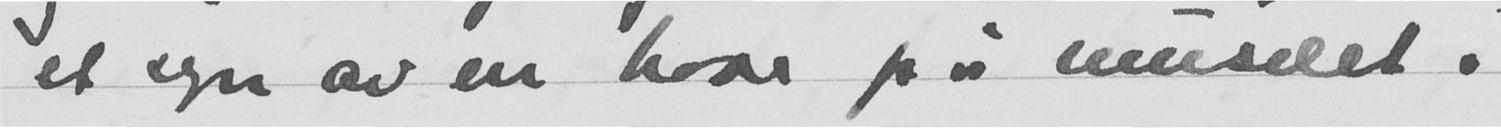et syn av en have på museet.
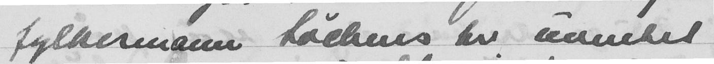fylkesmann Talknes kr uventet
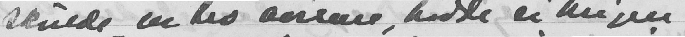skulde en tro avisene, hadde vi krigen
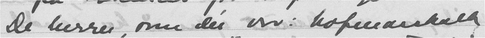De herrer, som der var; hofmarskalk
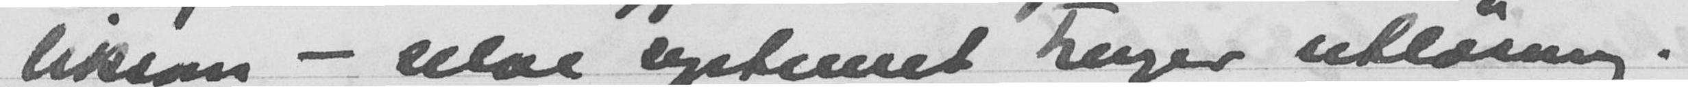liksom - selve systemet trenger utløsing.
